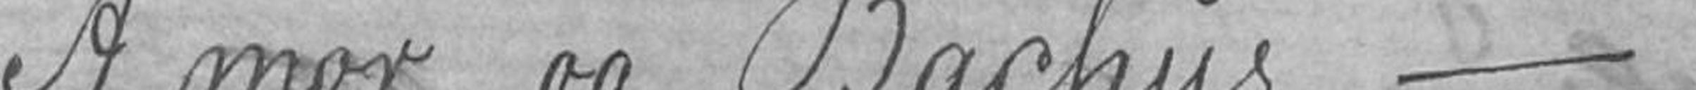A' mor og Bachus -
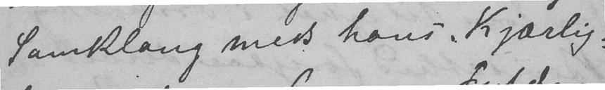Samklang med hans. Kjærlig-
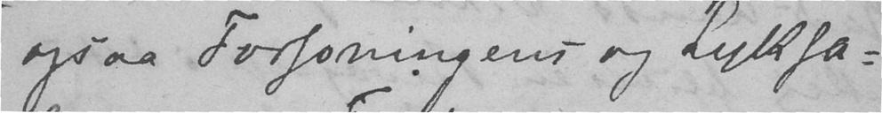ogsaa Forsoningens og Lyksa-
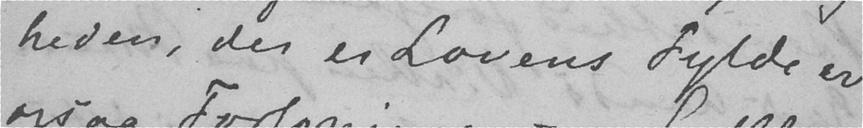heden, der er Lovens fylde er
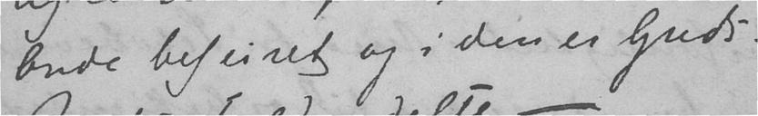Onde beseiret og i den er Guds-
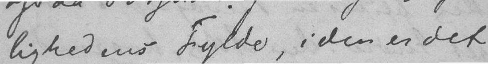lighedens Fylde, i den er det
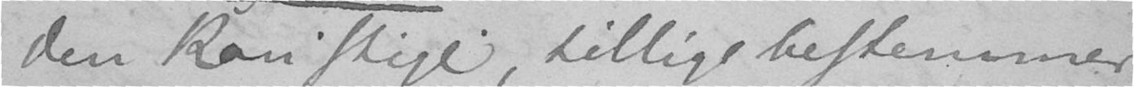den kan stige, tillige bestemmer
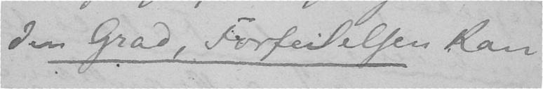den Grad, Forfeilelsen kan
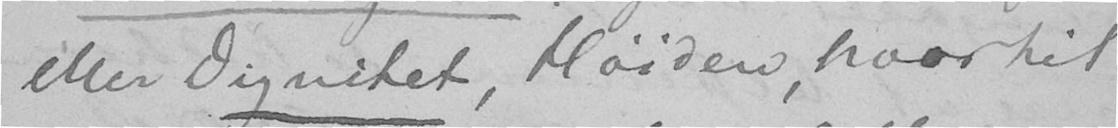eller Dignitet, Høiden, hvortil
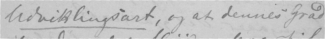Udviklingsart, og at dennes Grad
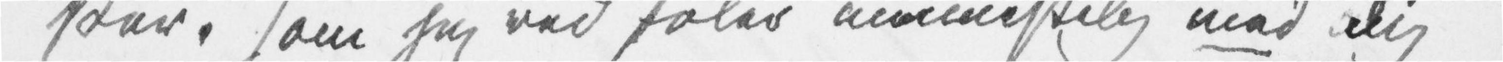sker, som jeg ved føler menneskelig med dig
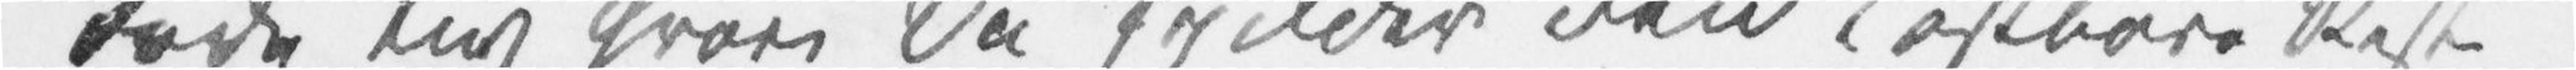døde Liv hvori Du spilder den kostbare Rest
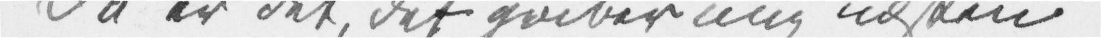Da er det, det griber mig næsten
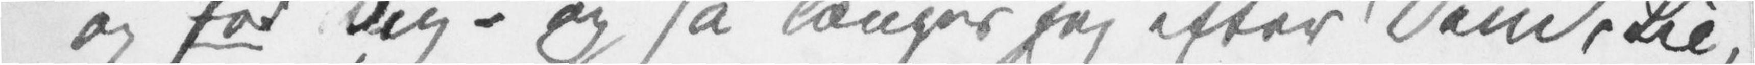og for dig – og så længes jeg efter Dem, Lie,
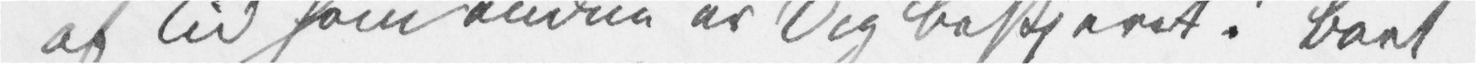af Tid som endnu er Dig beskjeret! Bort
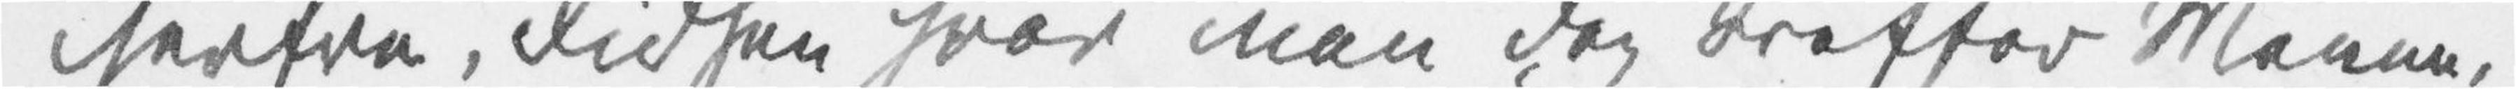herfra, didhen hvor man dog treffer Menne¬
